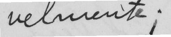velmente;
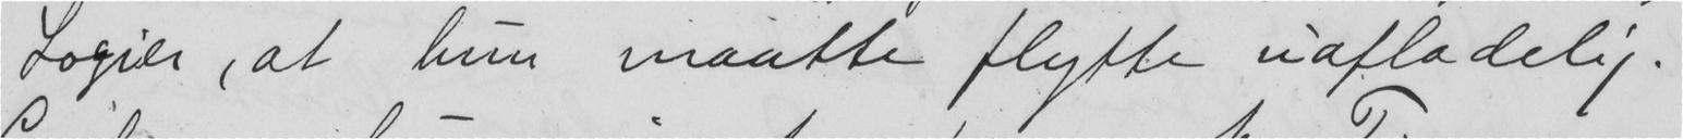Logier, at hun maatte flytte uafladelig.
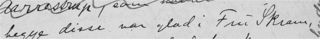begge disse var glad i Fru Skram,
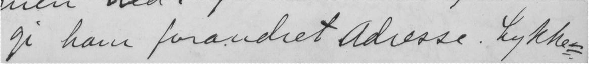gi ham forandret Adresse. Lykke-
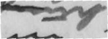hun
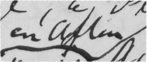en Aften
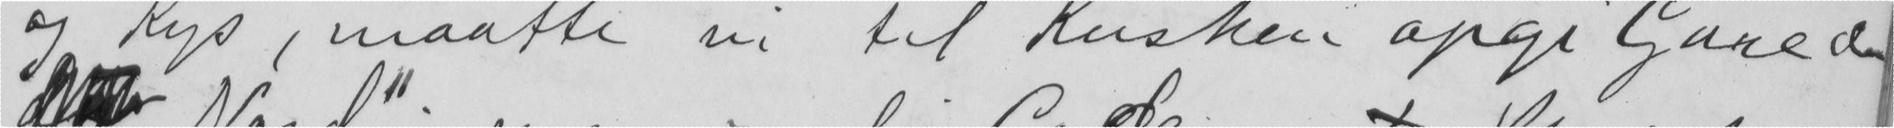og Kys, maatte vi til Kusken opge "Gare de
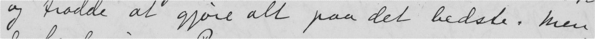og trodde at gjøre alt paa det bedste. Men
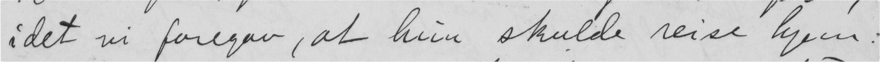idet vi foregav, at hun skulde reise hjem.
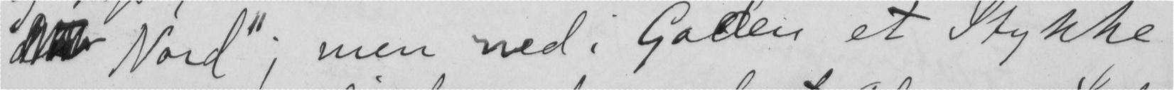Nord"; men ned i Gaden et stykke
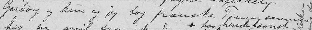Garborg og hun og jeg tog franske Timer samme
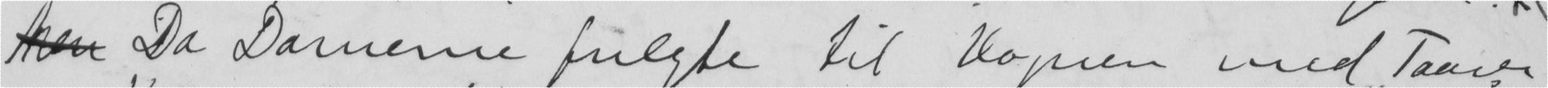Da Damerne fulgte til Vognen med Taarer
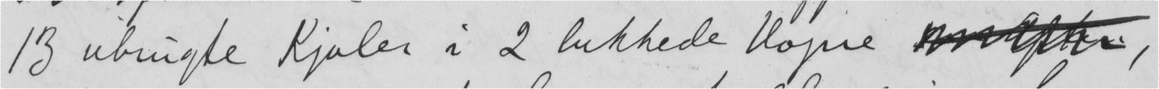13 ubrugte Kjoler i 2 lukkede Vogne ,
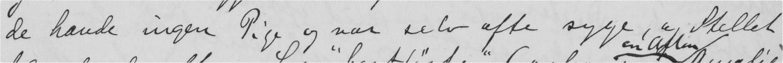de havde ingen Pige og var selv ofte syge og stellet
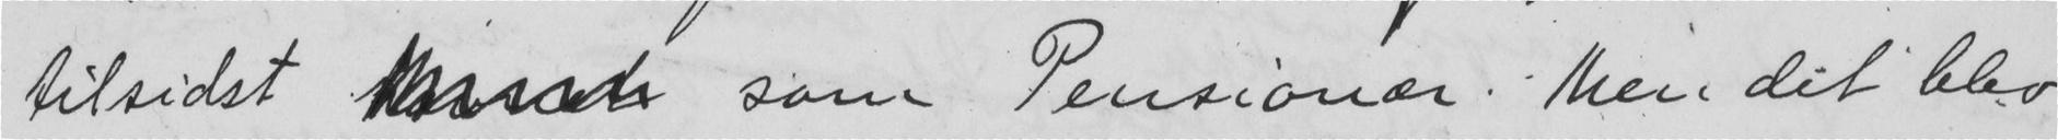tilsidst  som Pensionær. Men det blev
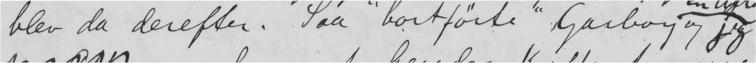blev da derefter. Saa "bortførte" Garborg og jeg
